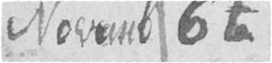Novemb 6te
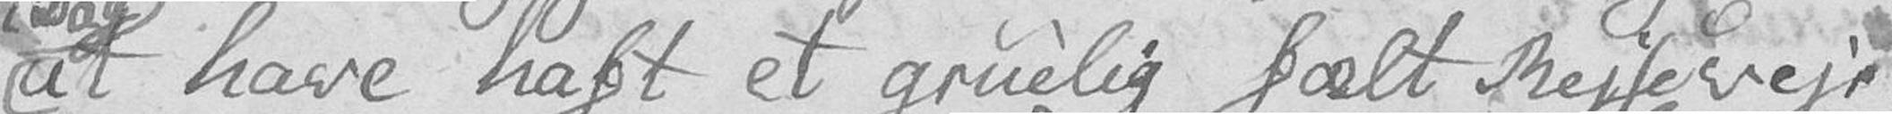i Dag at have haft et gruelig fælt Rejsevejr
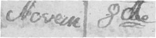Novem 8de
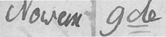Novem 9de
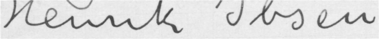Henrik Ibsen
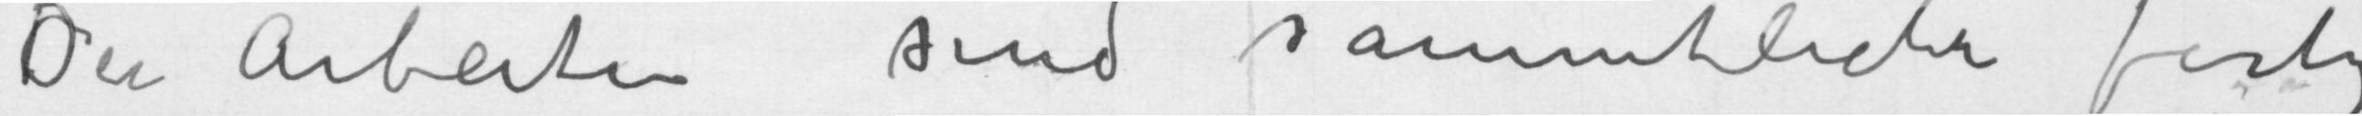Die Arbeiten sind sammtliche fertig
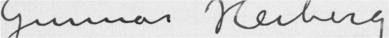Gunnar Heiberg
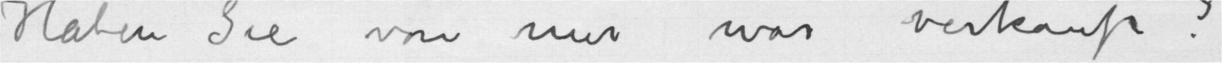Haben Sie von mir was verkauft?
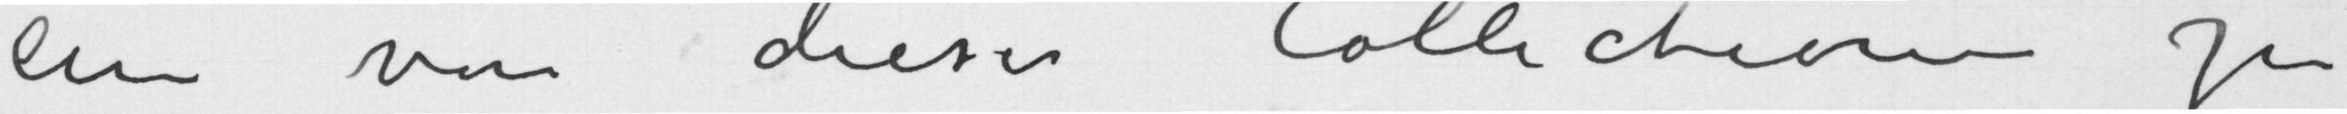ein von dieser Collection zu
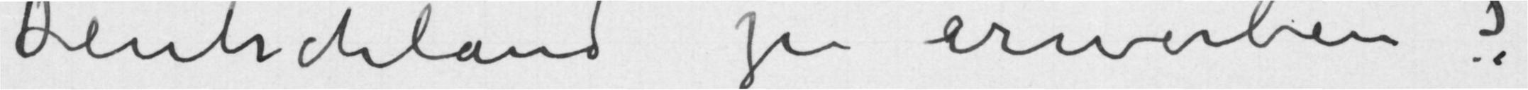Deutschland zu erwerben?
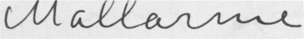Mallarmé
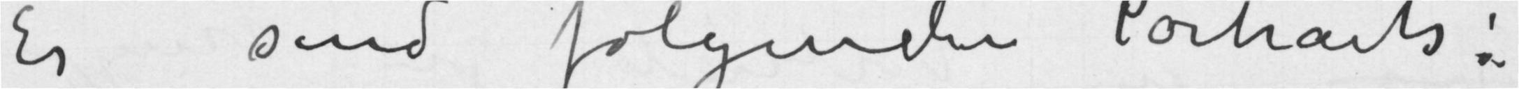Es sind folgender Portraits:
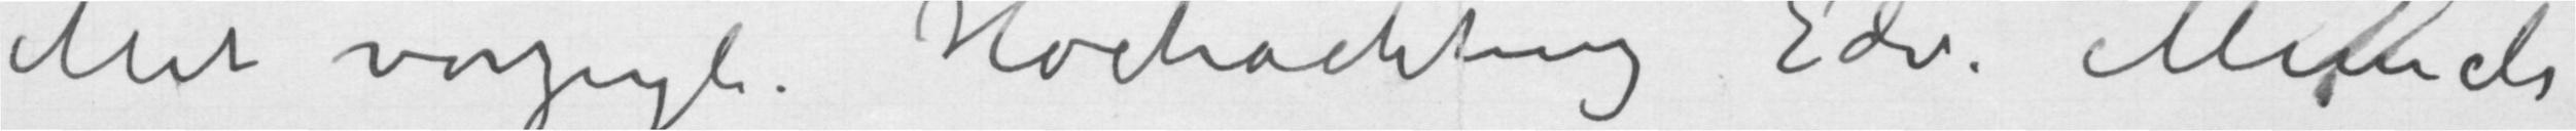Mit vorzugl. Hochachtung Edv. Munch
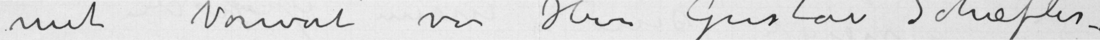mit Vorwort von Hrr Gustav Schiefler –
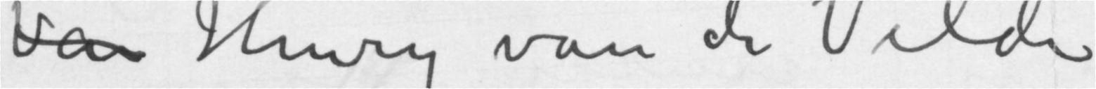van Henry van de Velde
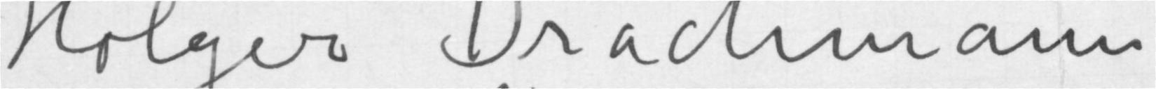Holger Drachmann
